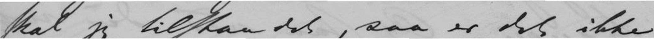skal jeg tilstaa det, saa er det ikke
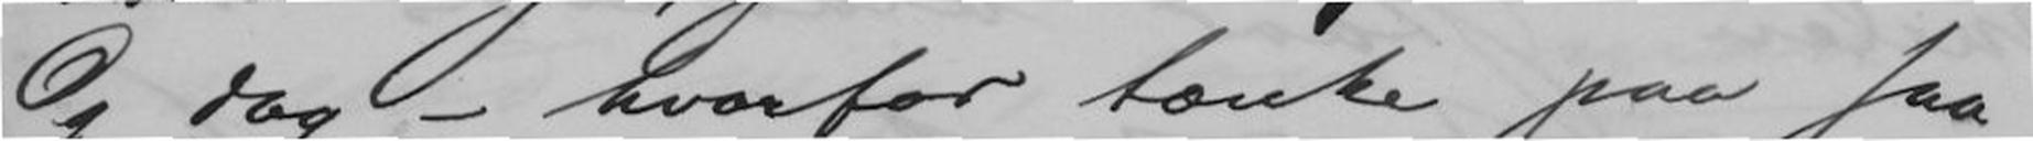Og dog - hvorfor tænke paa saa-
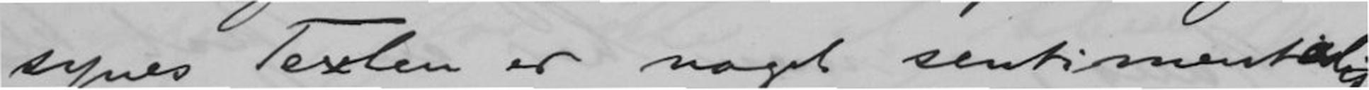synes Texten er noget sentimentalis-
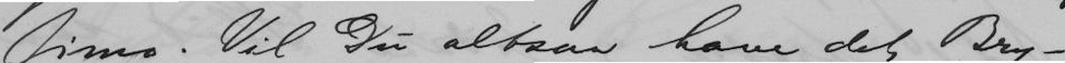simo. Vil Du altsaa have det Bry-
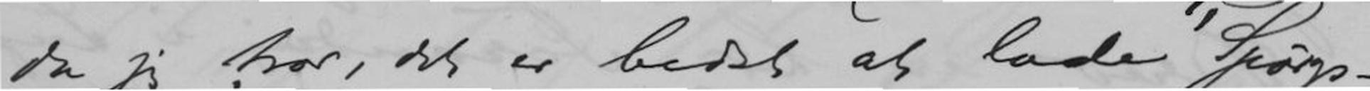da jeg tror, det er bedst at lade Spørgs-
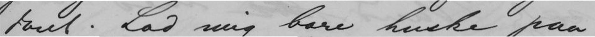dant. Lad mig bare huske paa
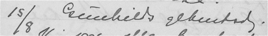15/8 Gunhilds geburtsdag.
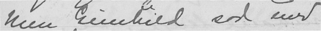Men Gunhild sad med
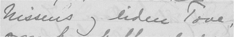Nissens og liden Tove,
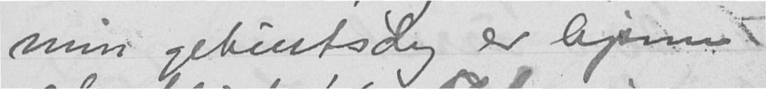min geburtsdag er ligsom
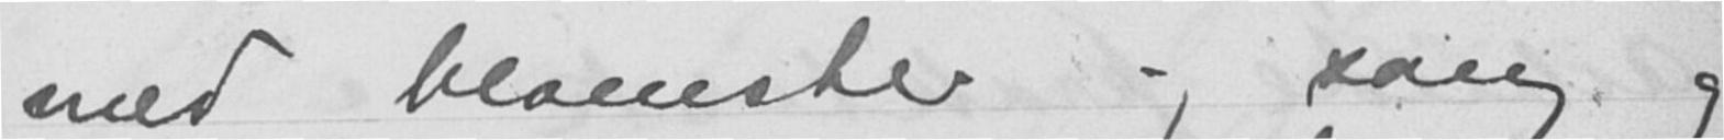med blomster og sang og
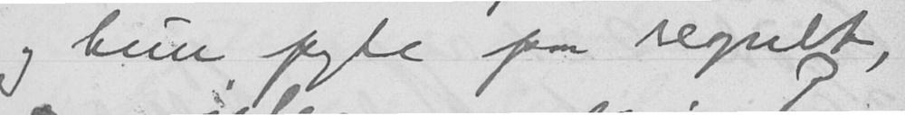og hun pegte paa regnet,
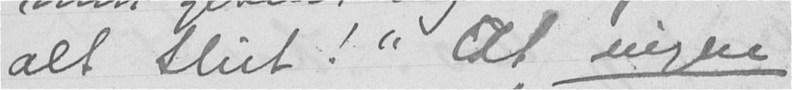alt slut!" At ingen
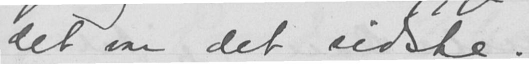det var det sidste.
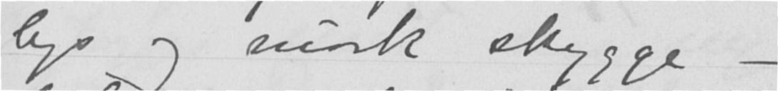lys og mørk skygge -
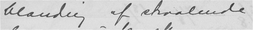blanding af straalende
